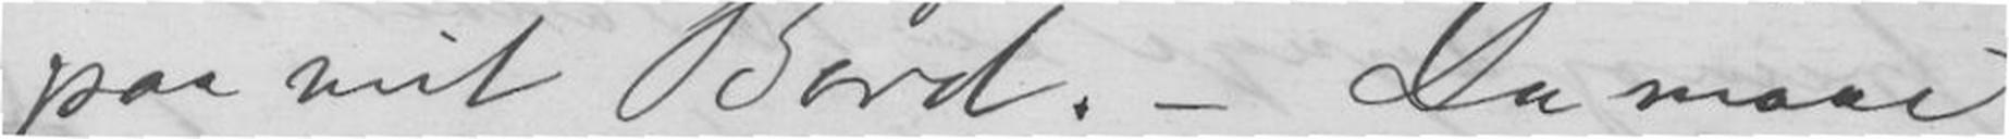paa mit Bord. - Du maae
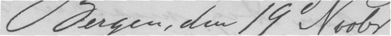Bergen, den 19d Novbr
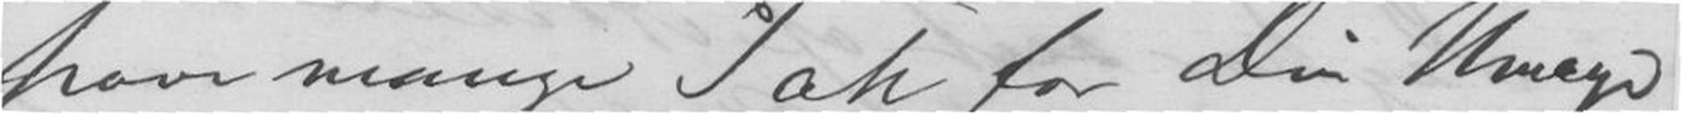have mangen Tak for Din Umage
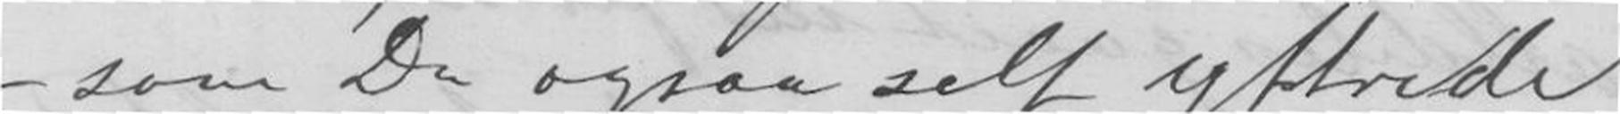- som Du ogsaa self yttrede
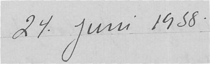24. juni 1938.
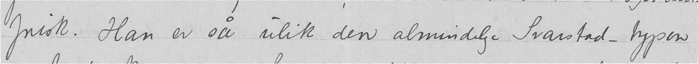frisk. Han er så ulik den almindelige Svarstad – typen
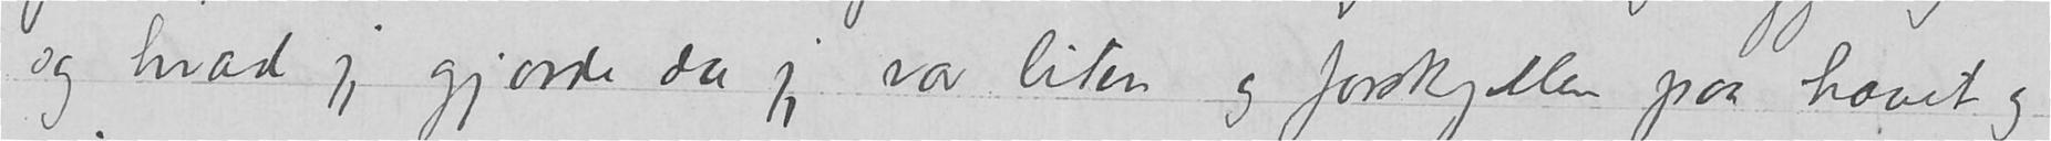og hvad jeg gjorde da jeg var liten og forskjellen paa havet og
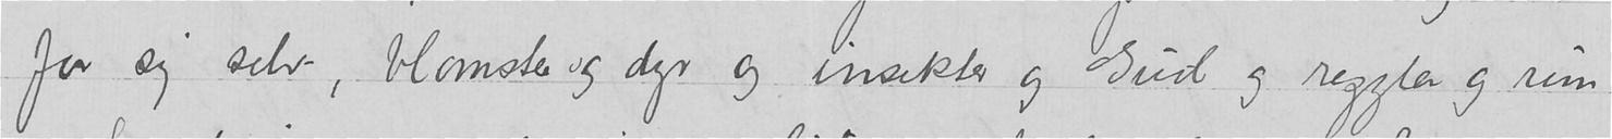for sig selv, blomster og dyr og insikter og Gud og reggler og rumn
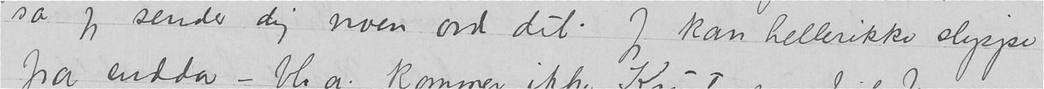så jeg sender dig noen ord dit. Jeg kan hellerikke slippe
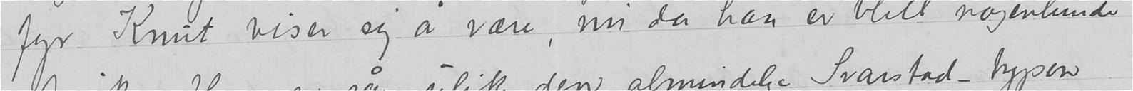fyr Knut viser sig av være, nu da han er blitt nogenlunde
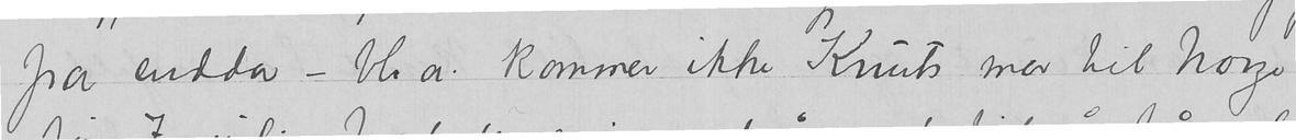fra endda – bl.a. kommer ikke Knuts mer til Norge
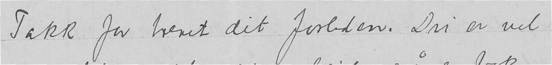Takk for brevet dit forleden. Du er vel
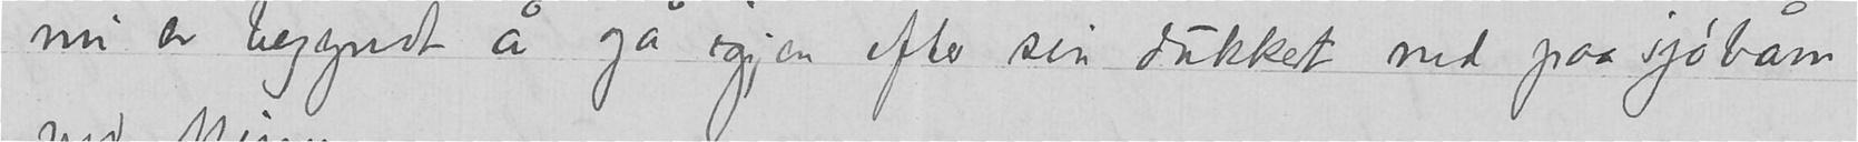nu er begyndt å gå igjen efter sin dukkert ned paa sjøbarn
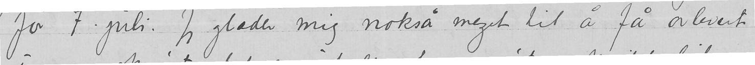før 7. juli. Jeg glæder mig noksa meget til å få avlevert
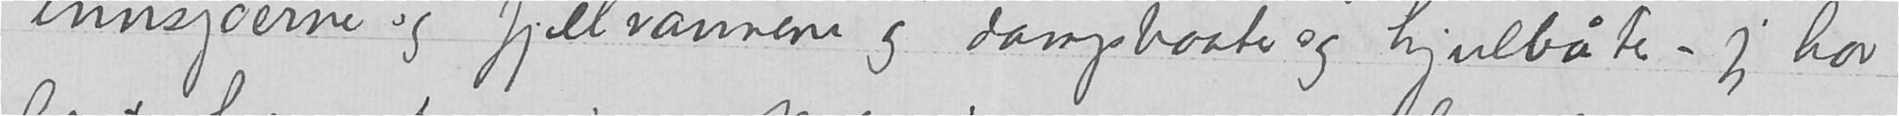innsjøerne og fjellvannene og dampbaater og hjulbåter – jeg har
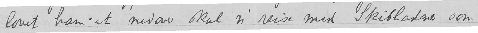lovet ham at nedover skal vi reise med Skibladner, som
